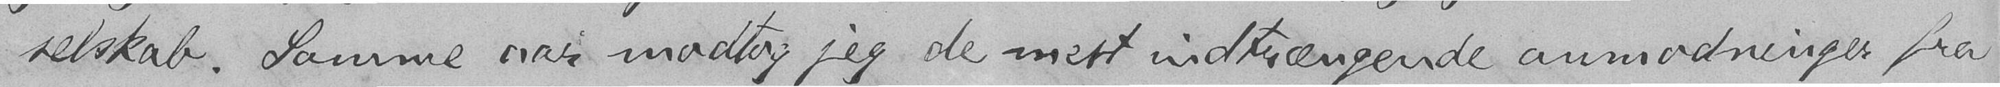selskab. Samme aar modtog jeg de mest indtrængende anmodninger fra
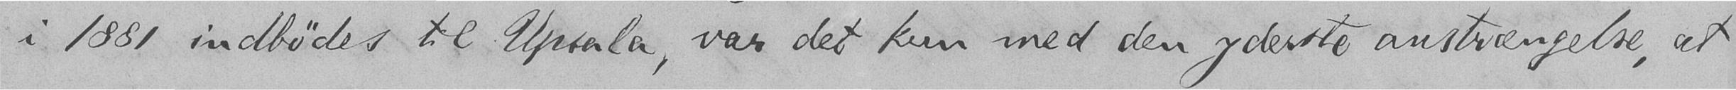i 1881 indbødes til Upsala, var det kun med den yderste anstrængelse, at
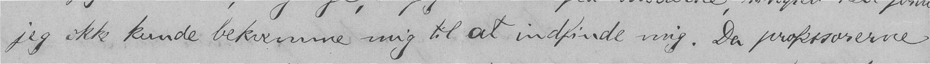jeg ikke kunde bekvemme mig til at indfinde mig. Da professorerne
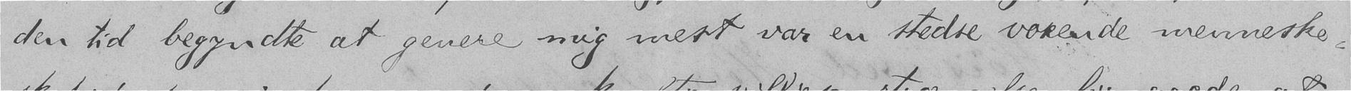den tid begyndte at genere mig mest var en stedse voxende menneske-
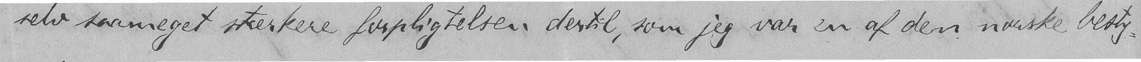selv saameget stærkere forpligtelsen dertil, som jeg var en af den norske besty-
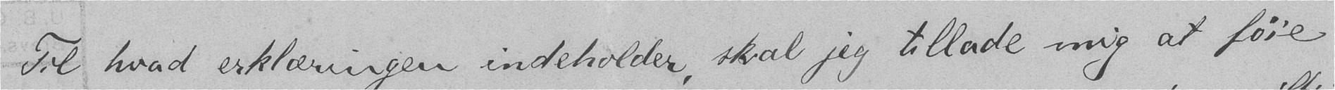Til hvad erklæringen indeholder, skal jeg tillade mig at føie
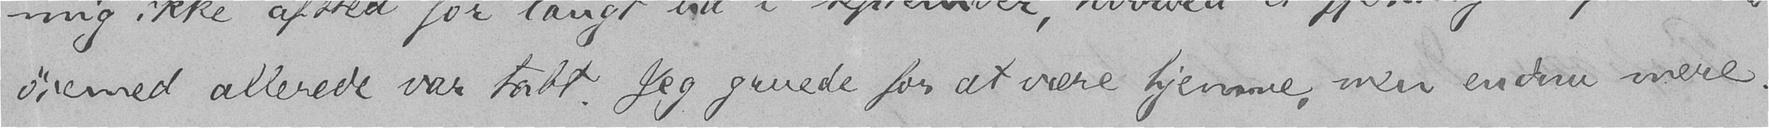øiemed allerede var tabt. Jeg gruede for at være hjemme, men endnu mere
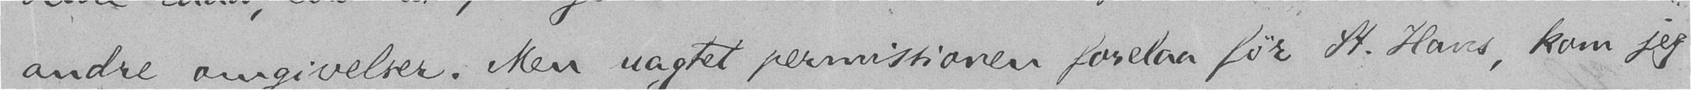andre omgivelser. Men uagtet permissionen forelaa før St. Hans, kom jeg
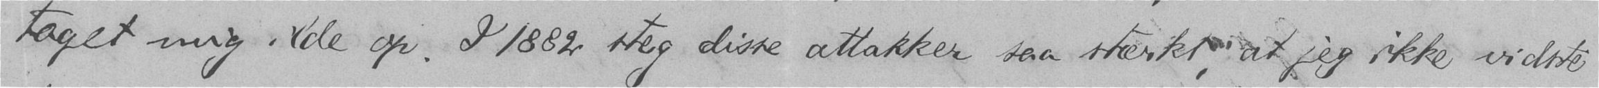taget mig ilde op. I 1882 steg disse attakker saa stærkt, at jeg ikke vidste
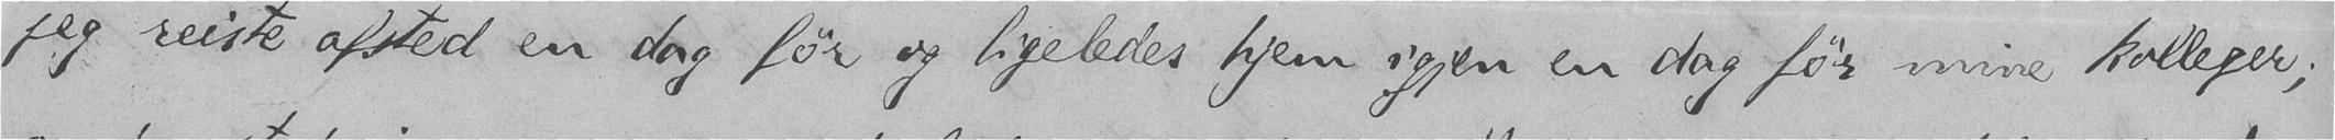jeg reiste afsted en dag før og ligeledes hjem igjen en dag før mine kolleger;
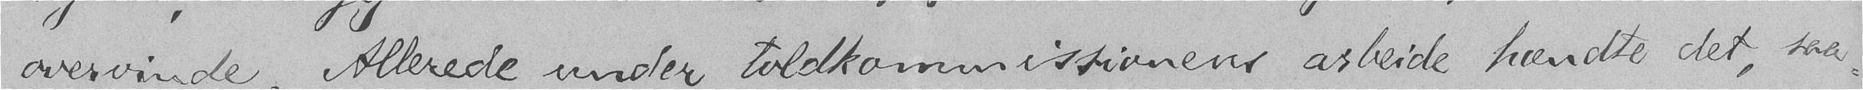overvinde. Allerede under toldkommissionens arbeide hændte det, saa-
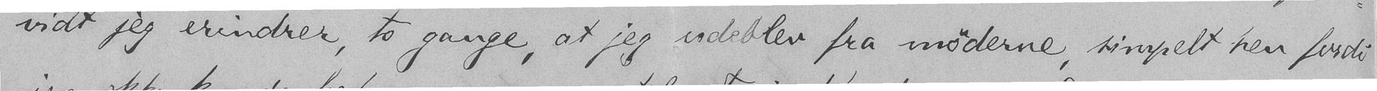vidt jeg erindrer, to gange, at jeg udeblev fra møderne, simpelt hen fordi
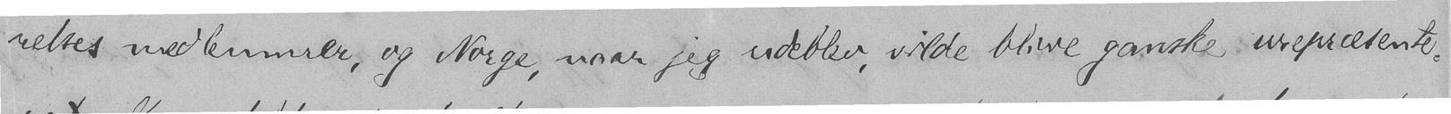relses medlemmer, og Norge, naar jeg udeblev, vilde blive ganske urepræsente-
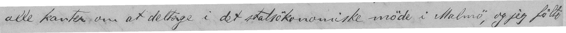alle kanter om at deltage i det statsøkonomiske møde i Malmö, og jeg følte
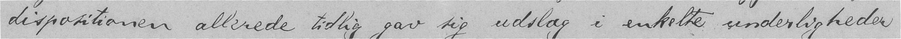dispositionen allerede tidlig gav sig udslag i enkelte underligheder
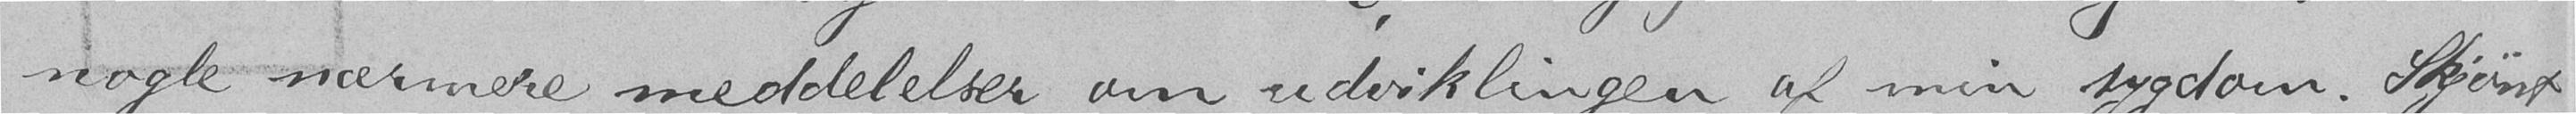nogle nærmere meddelelser om udviklingen af min sygdom. Skjønt
